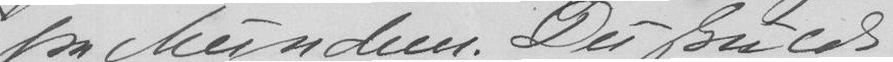fra München. Du skulde
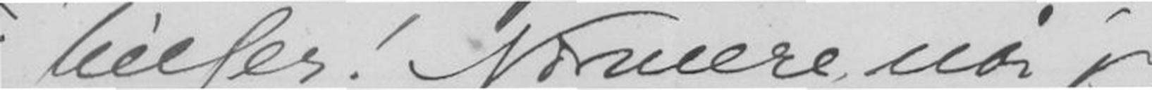hilser! Nærmere når jeg
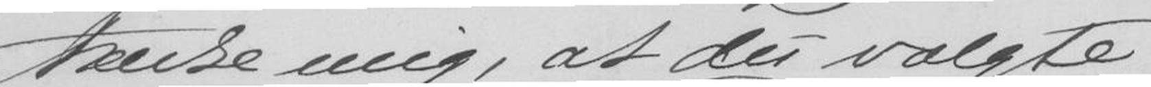tænke mig, at du valgte
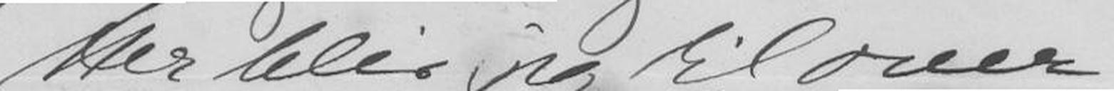Her blir jeg til over
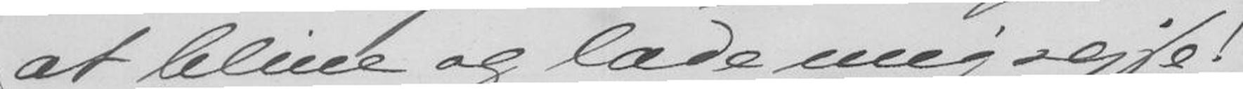at blive og lade mig rejse!
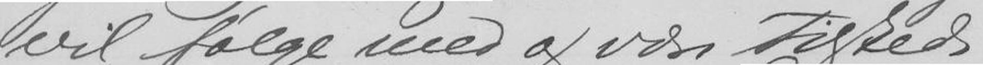vil følge med og være tilstede
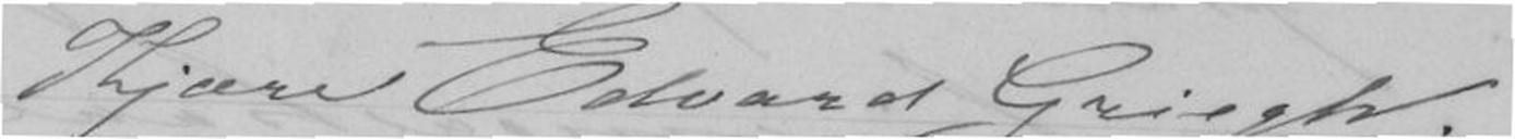Kjære Edvard Griegh.
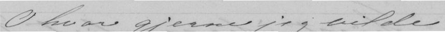O hvor gjerne jeg vilde
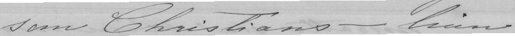som Christians - hun
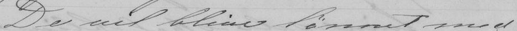De vil blive lønnet med
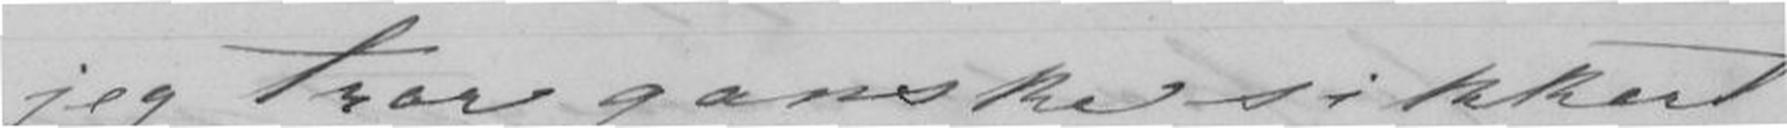jeg tror ganske sikkert
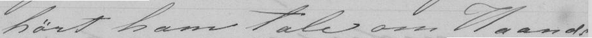hørt ham tale om Haand-
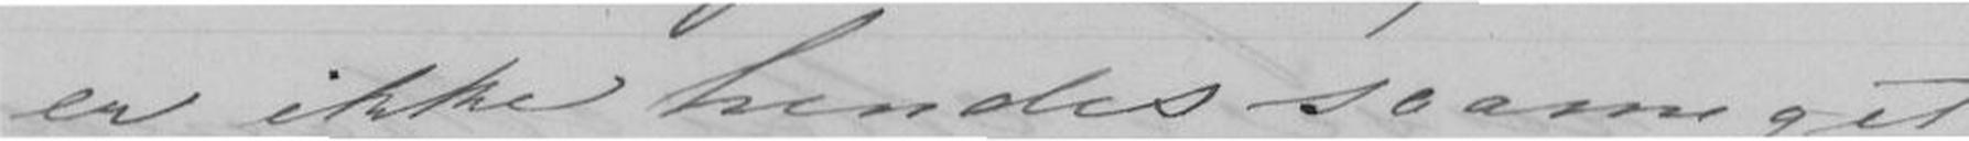er ikke hendes saameget
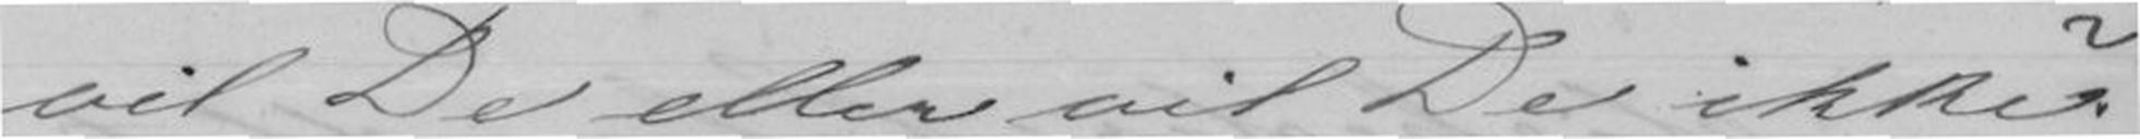vil De eller vil De ikke?
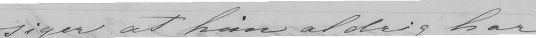siger at hun aldrig har
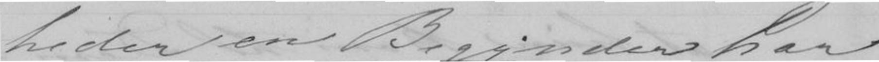heder en Begynder har
